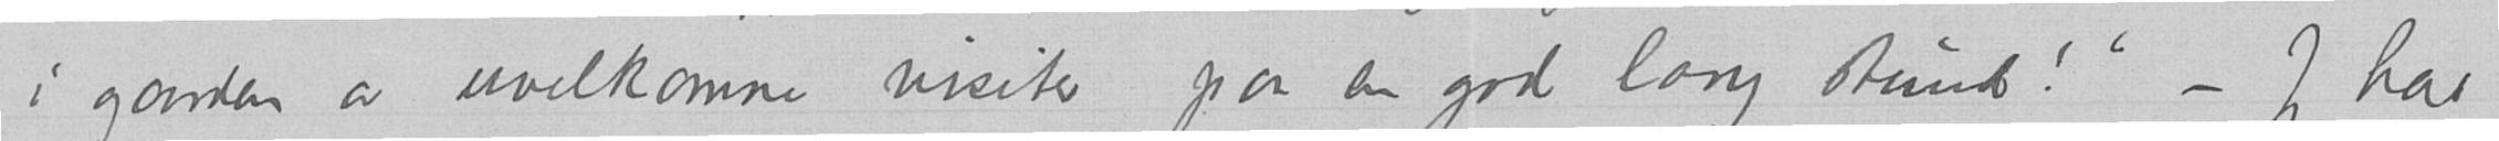i gaarden av uvelkomne visiter paa en god lang stund!" - Jeg har
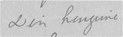Din hengivne
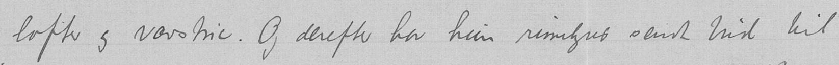lofter og værstue. Og derefter har hun rimeligst sendt bud til
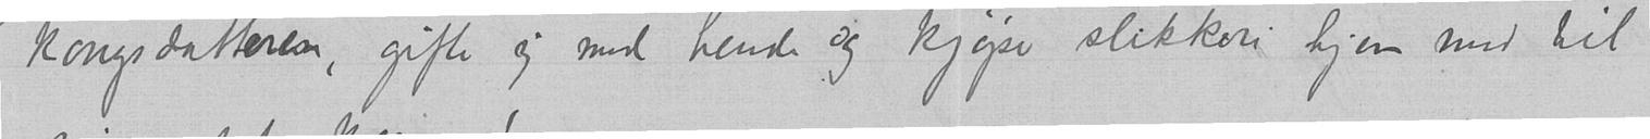kongsdatteren, gifter sig med hende og kjøper slikkeri hjem med til
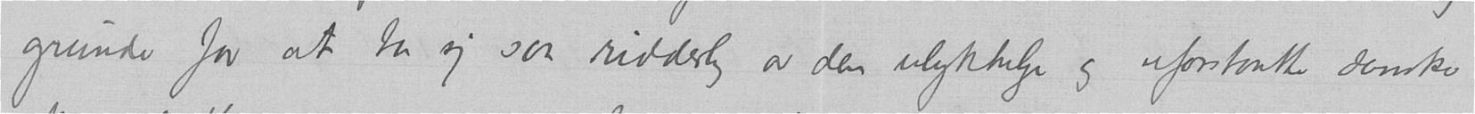grunde for at ta sig saa ridderlig av den ulykkelige og uforstaatte danske
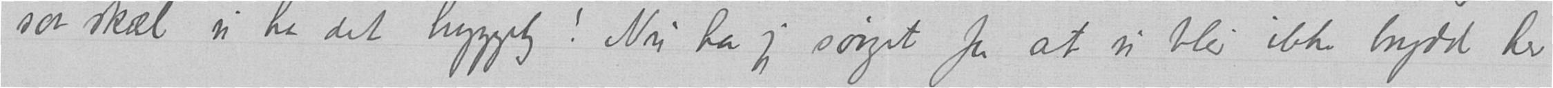saa skal vi ha det hyggelig! Nu har jeg sørget for at vi blir ikke brydd her
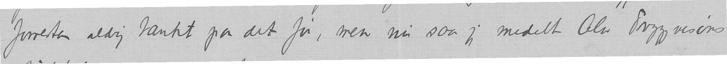forresten aldrig tænkt paa det før, men nu saa jeg medett Olav Trygvesøns
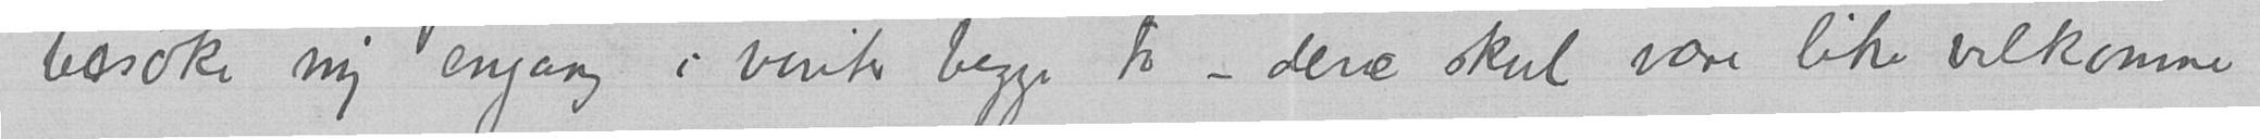besøke mig engang i vinter begge to – dere skal være like velkomme
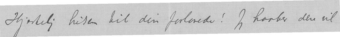Hjertelig hilsen til din forlovede! Jeg haaber dere vil
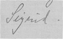Sigrid.
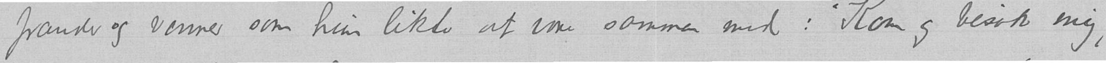frænder og venner som hun likte at være sammen med: "Kom og besøk mig,
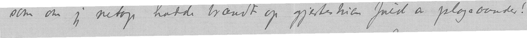som om jeg netop hadde brændt op gjestestuen fuld av plageaander!
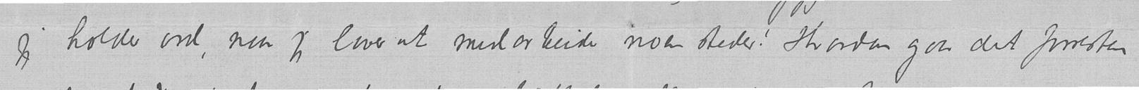jeg holder ord, naar jeg lover at medarbeide noen steder! Hvordan gaar det forresten
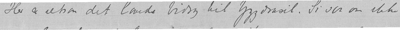Her er altsaa det lovede bidrag til Yggdrasil. Si saa om ikke
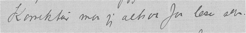Korrektur maa jeg altsaa faa lese selv.
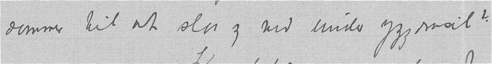dommer til at slaa sig ned under yggdrasil?
